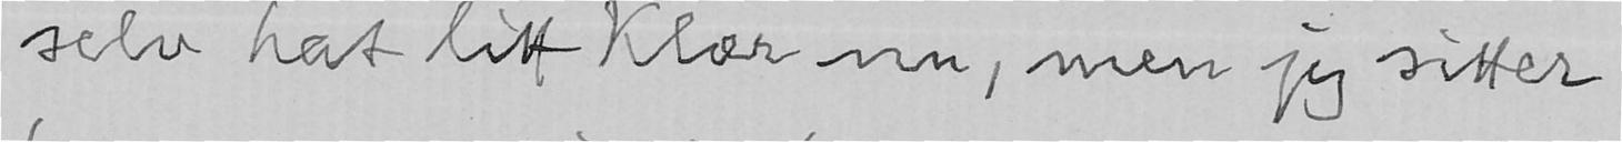selv hat litt Klær nu, men jeg sitter
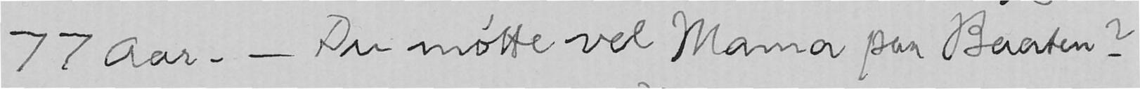77 Aar. - Du møtte vel Mama paa Baaten?
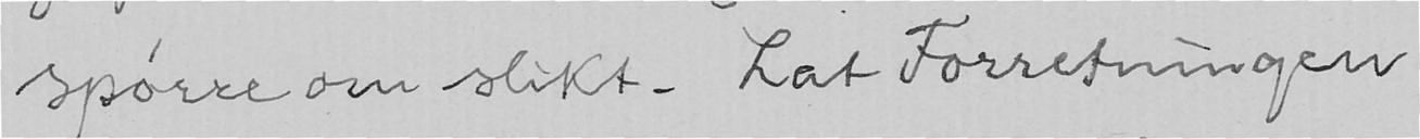spørre om slikt. Lat Forretningen
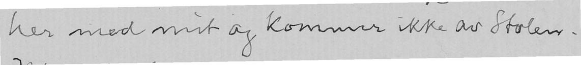her med mit og kommer ikke av Stolen.
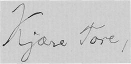Kjære Tore,
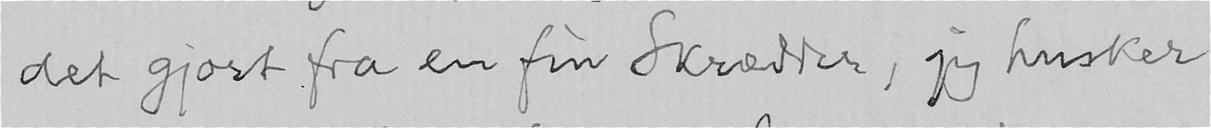det gjort fra en fin Skrædder, jeg husker
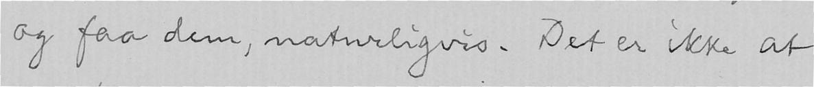og faa dem, naturligvis. Det er ikke at
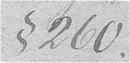§ 260.
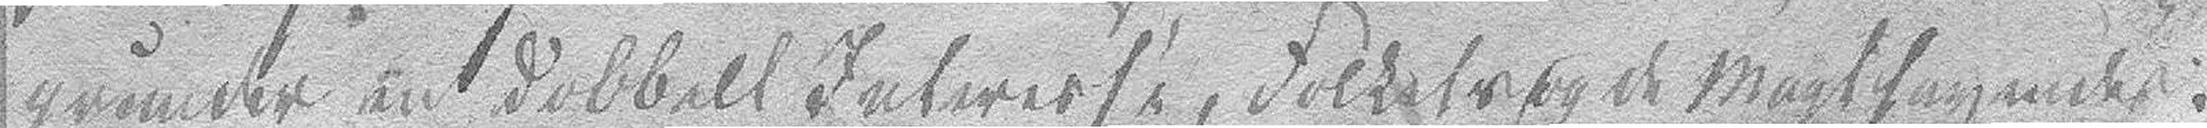grunder en dobbelt Interesse, Folkets og de Magthavendes.
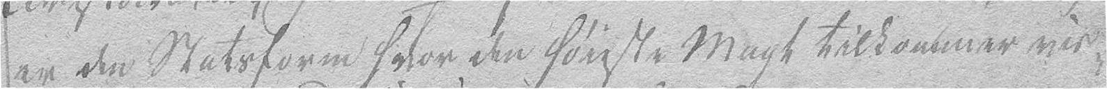er den Statsform hvor den høieste Magt tilkommer vis-
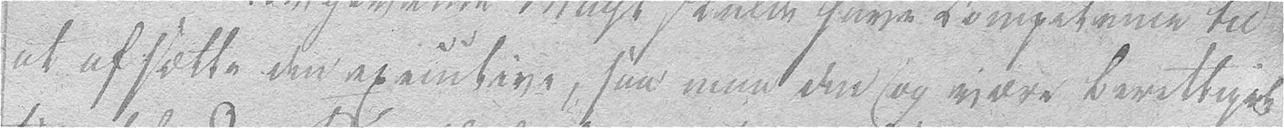at afsætte den executive, saa maatte den og være berettiget
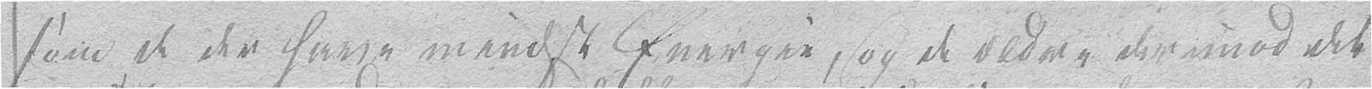som de der have mindst Energie, og de andre derimod det
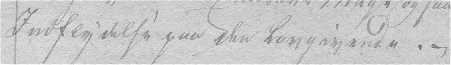Indflydelse paa den Lovgivende. –
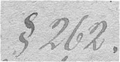§ 262.
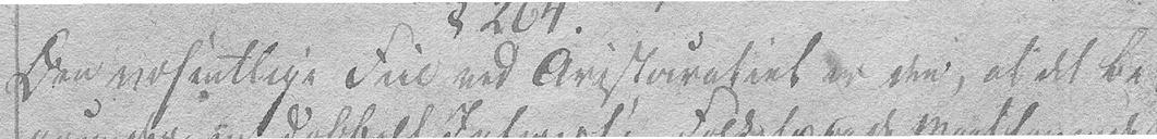Den væsentlige Feil ved Aristocratiet er den, at det be-
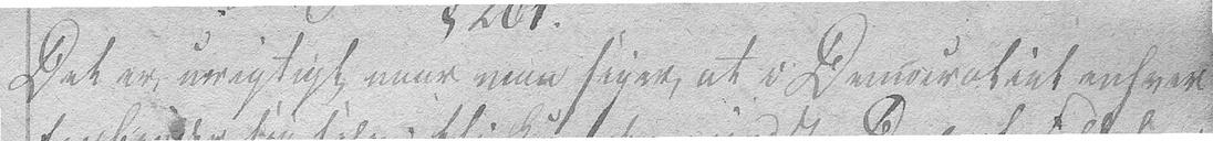Det er urigtigt naar man siger, at i Democratiet enhver
